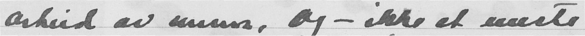arbeid av menn, Og - ikke et eneste
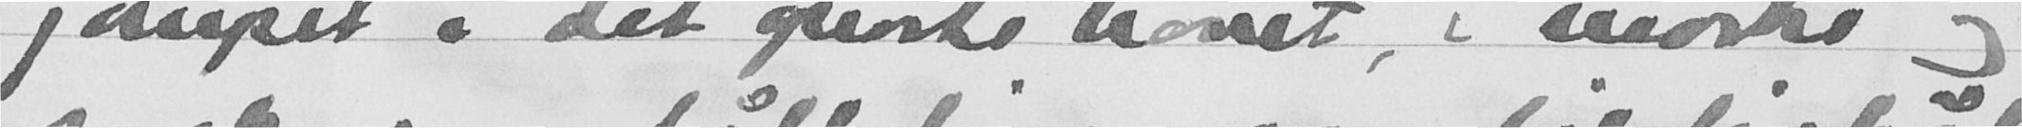jumpet i det oprørte havet, i mørke og
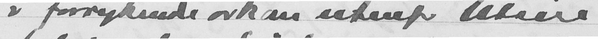i forrykende orkan utenfor Utsira
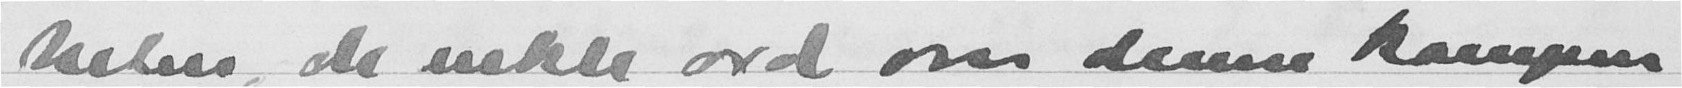heten, de enkle ord om denne kampen
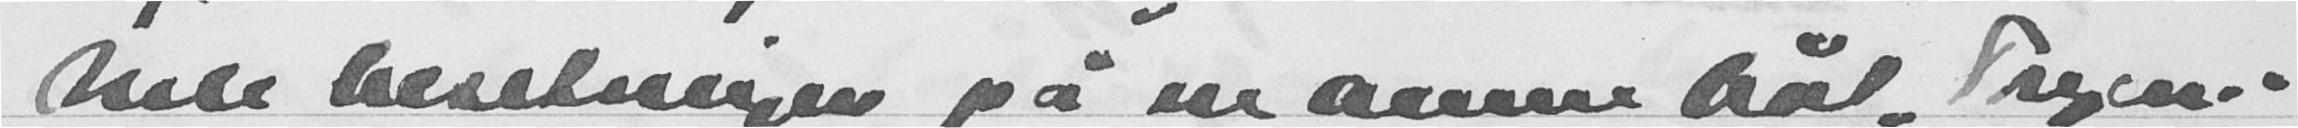hele besetningen på en annen båt "Fryen"
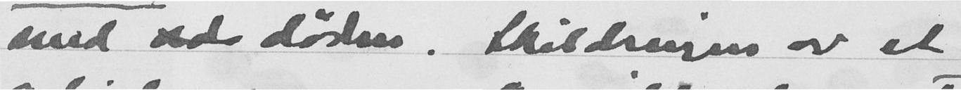med  døden. Skildringen av et
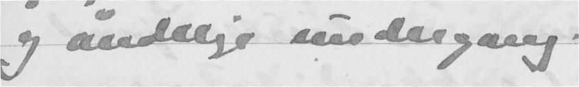og åndelige undergang.
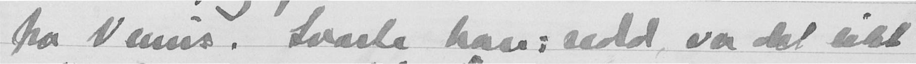fra Veums. Svarte han: redd va det likt
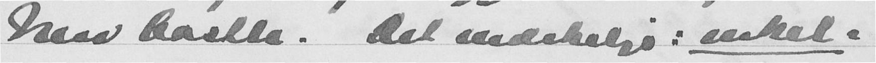New Castle. Det merkelige: enkel-
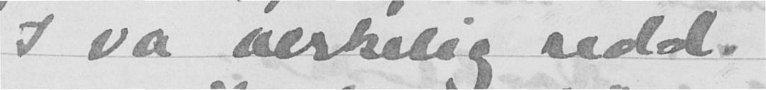I va verkelig redd.
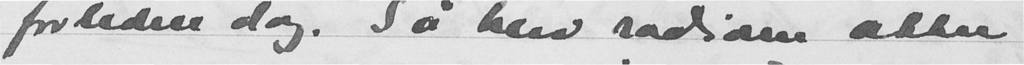forleden dag. Så blev radioen atter
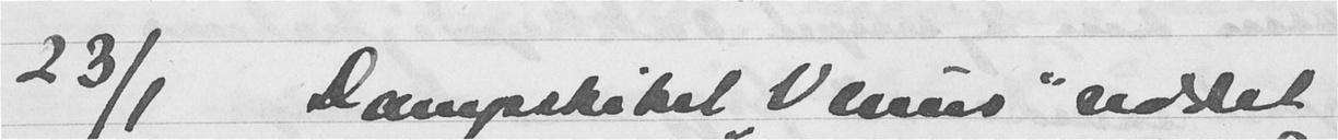23/1 Dampskibet "Veum" reddet
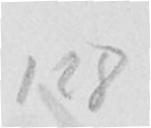128
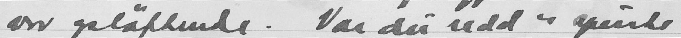var opløftende. Var du redd" spurte
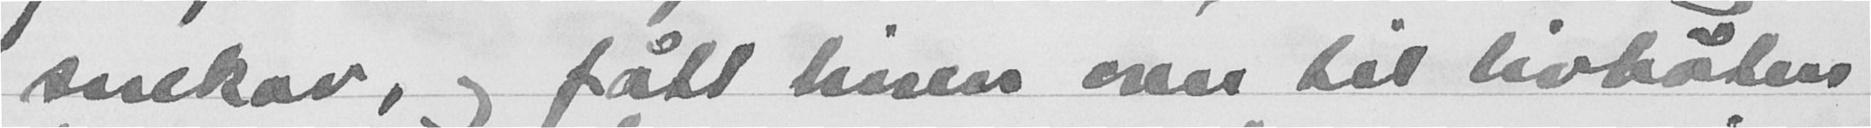snekav, og fått hiuen over til livbåten
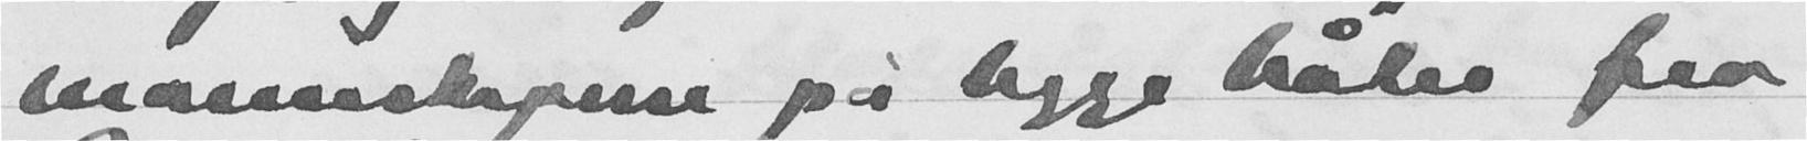mannskapene på begge båter fra
